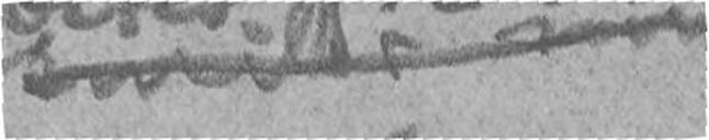smiler nu
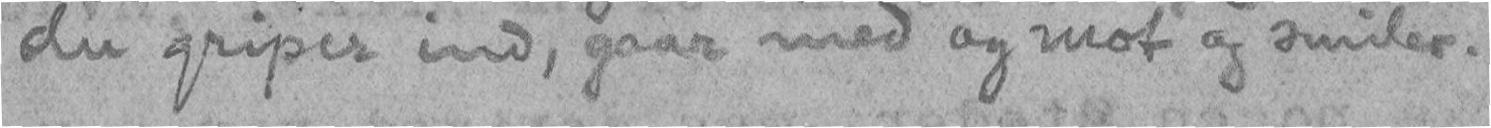du griper ind, gaar med og mot og smiler.
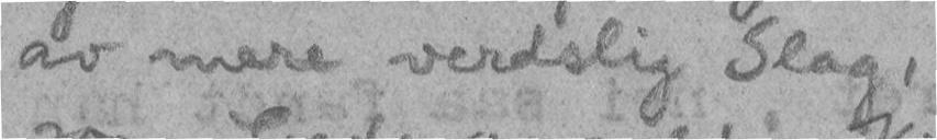av mere verdslig Slag,
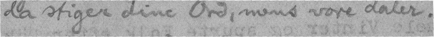da stiger dine Ord, mens vore daler.
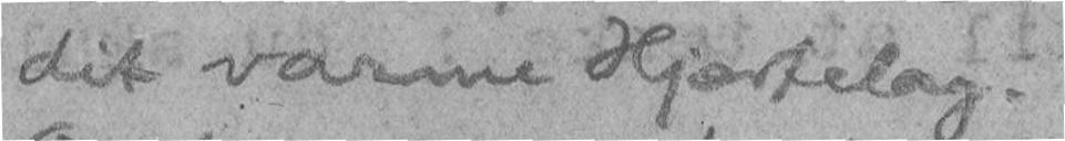dit varme Hjærtelag.
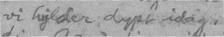vi hylder dypt idag,
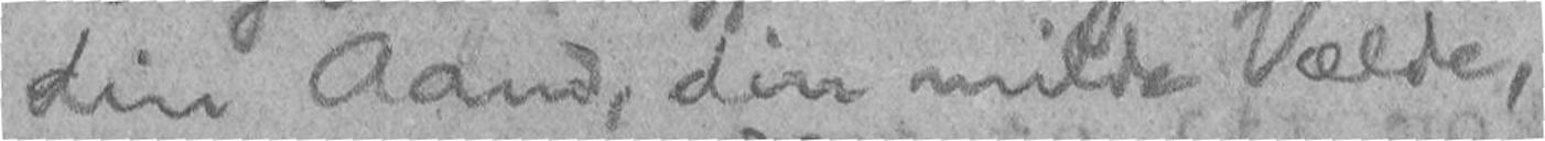din Aand, din milde Vælde,
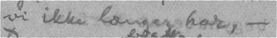vi ikke længer har, -
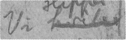Vi hariled
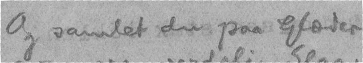Og samlet du paa Glæder
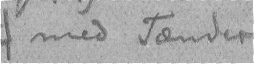med Tænder
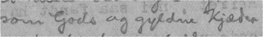som Gods og gyldne Kjæder
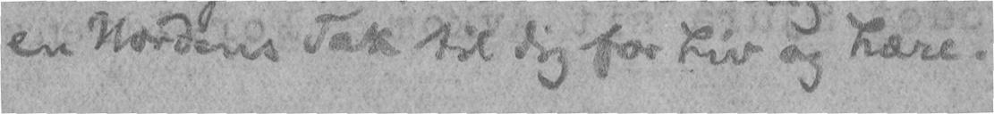en Nordens Tak til dig for Liv og Lære.
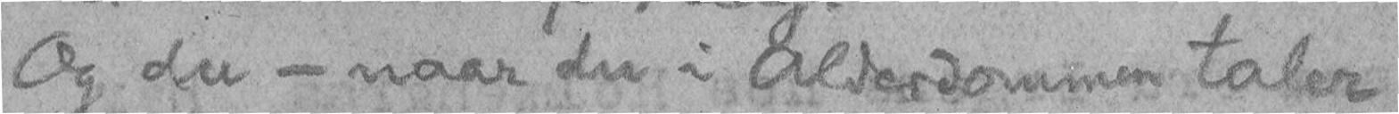Og du - naar du i Alderdommen taler
